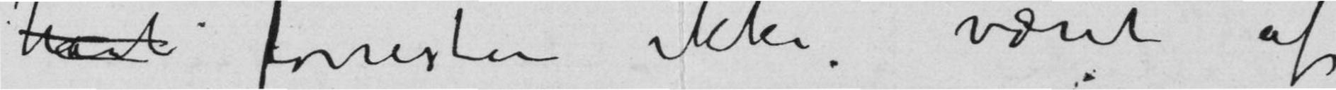havt forresten ikke været af
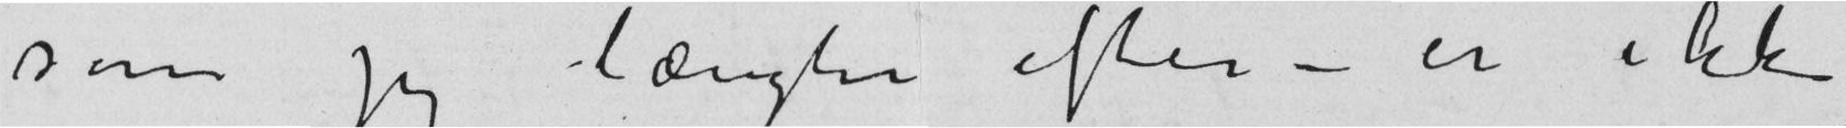som jeg længter efter – er ikke
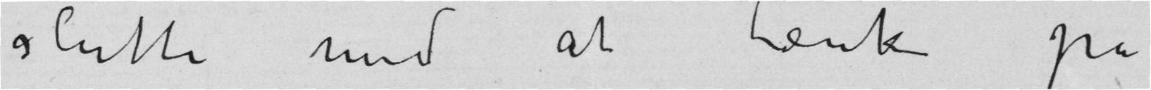slutte med at tænke på
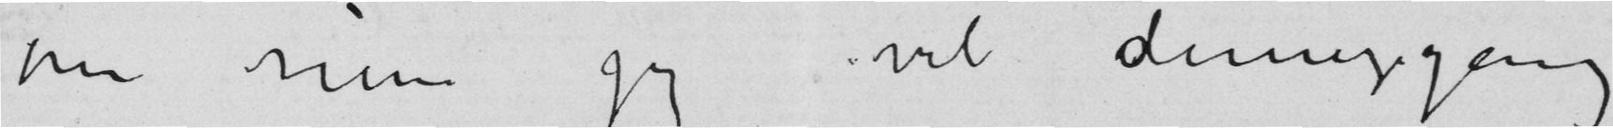om men jeg vil dennegang
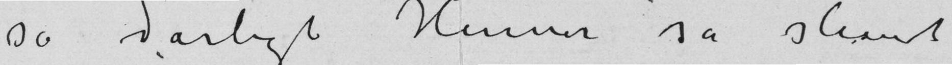så dårligt Humør så slemt
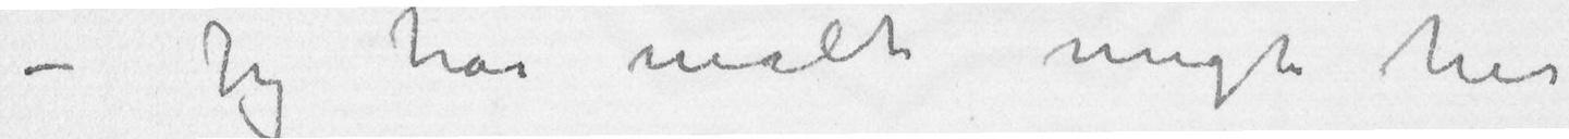– Jeg har malt meget her
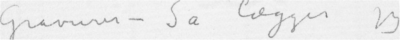Gravurer – Så lægger jeg
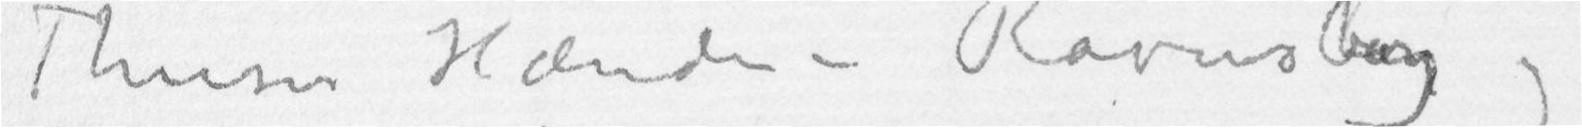Thiises Hænder – Ravensberg og
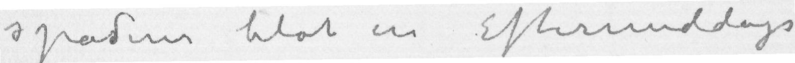spadserer blot en Eftermiddags
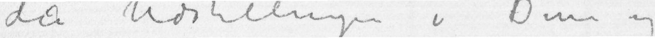da Udstillingen i Dine og
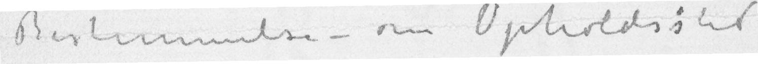Bestemmelse – om Opholdssted
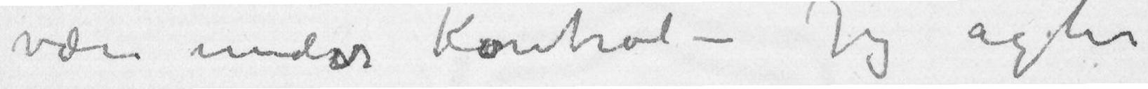være under Kontrol – Jeg agter
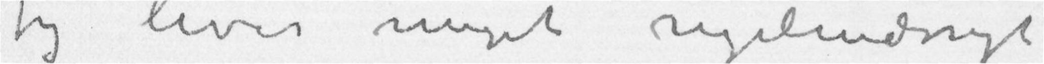Jeg lever meget regelmæssigt
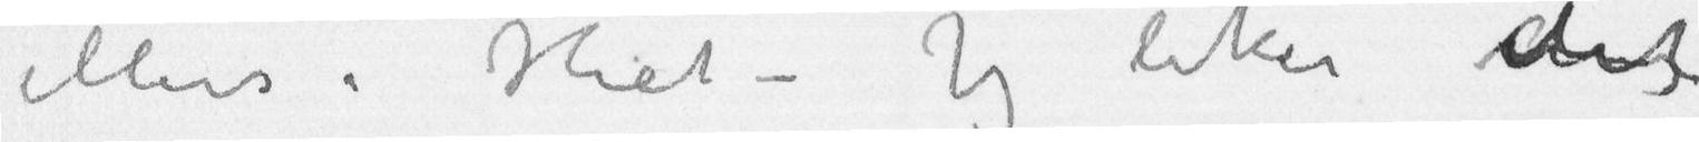ellers i Hiet – Jeg liker det
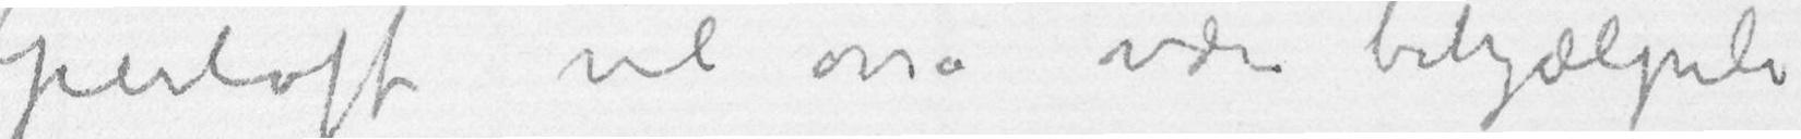Gierløff vil osså være behjælpeli
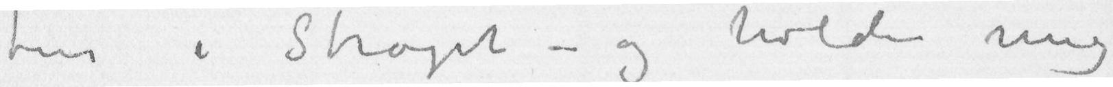tur i Strøget – og holder mig
